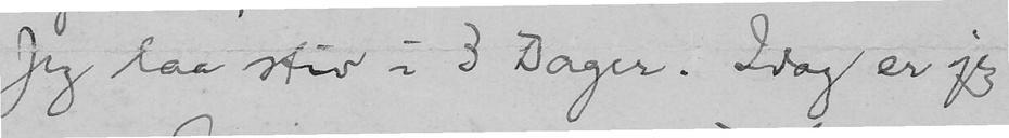Jeg laa stiv i 3 Dager. Idag er jeg
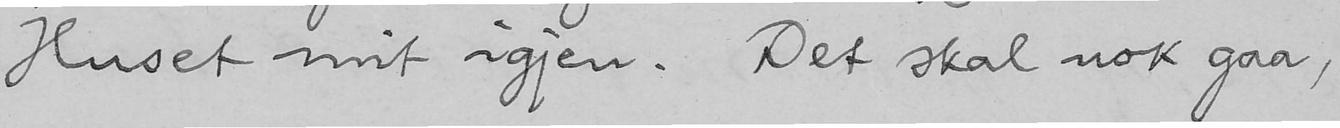Huset mit igjen. Det skal nok gaa,
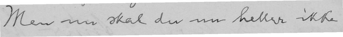Men nu skal du nu heller ikke
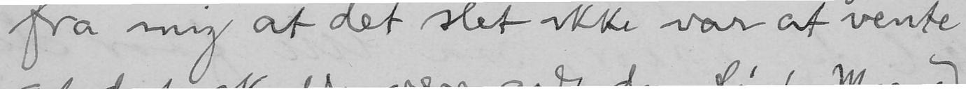fra mig at det slet ikke var at vente
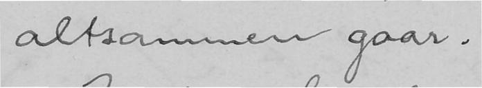altsammen gaar.
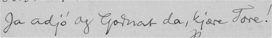Ja adjø og Godnat da, kjære Tore!
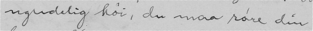ugudelig høi, du maa røre din
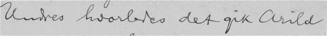Undres hvorledes det gik Arild
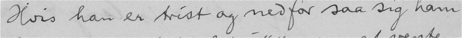Hvis han er trist og nedfor saa sig ham
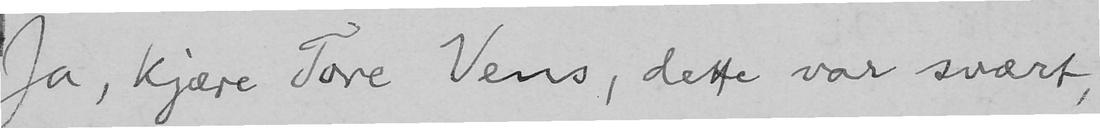Ja, kjære Tore Vens, dette var svært,
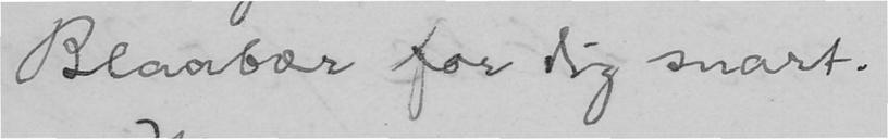Blaabær for dig snart.
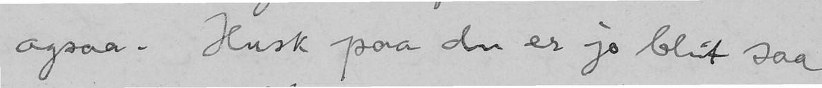ogsaa. Husk paa du er jo blit saa
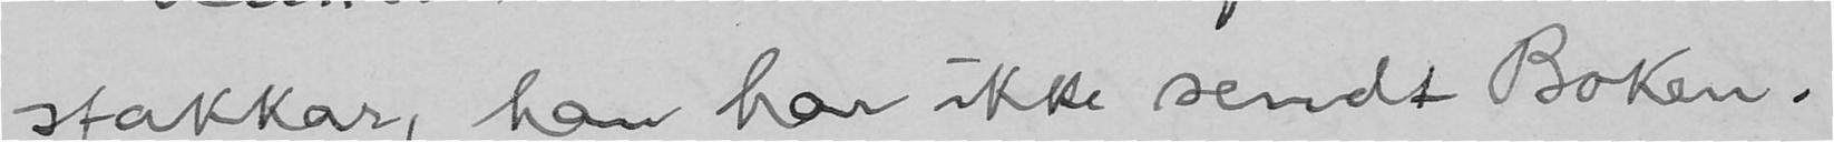stakkar, han har ikke sendt Boken.
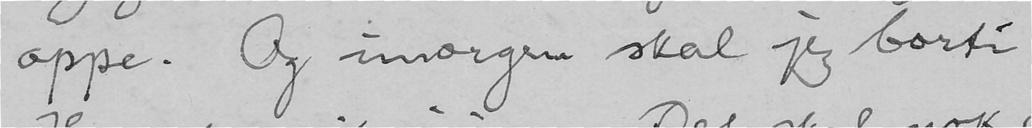oppe. Og imorgen skal jeg borti
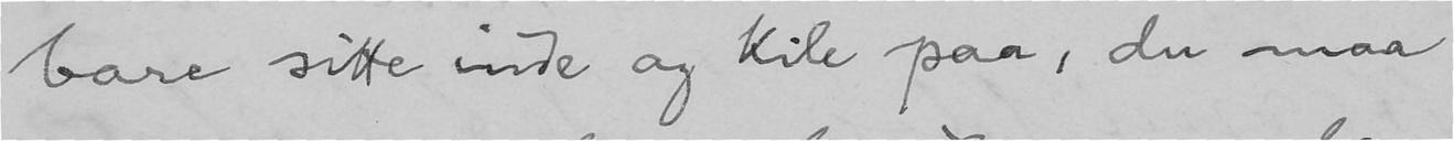bare sitte inde og kile paa, du maa
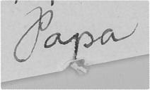Papa
